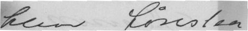først maa blive foreslaa-
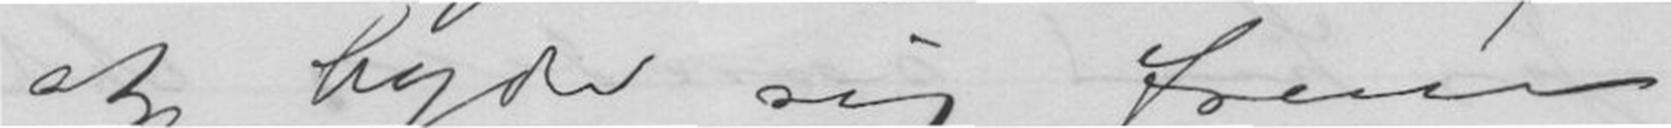at byde sig frem
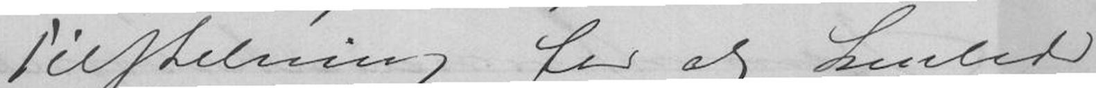Tilstelning for at henlede
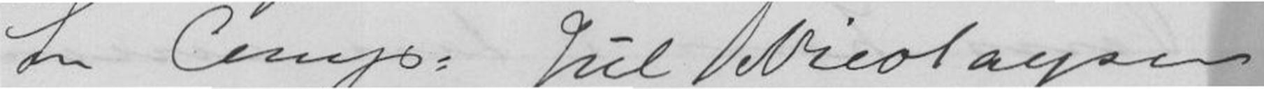til Comp: Jul Nicolaysen
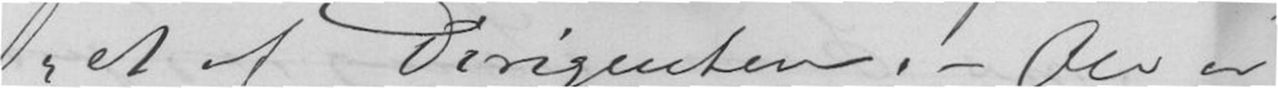et af Dirigenten. - Ole er
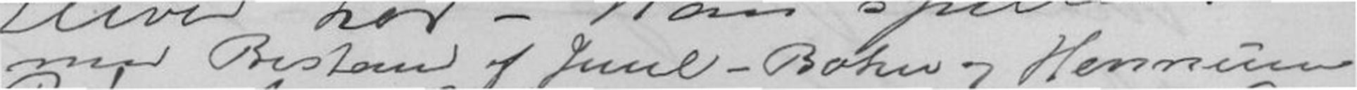Prøvelokalet
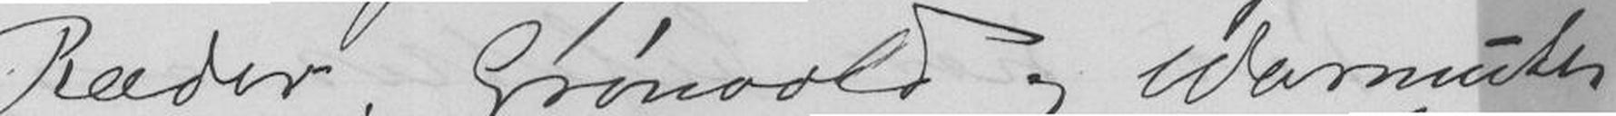Ræder, Grønvold og Warmuth
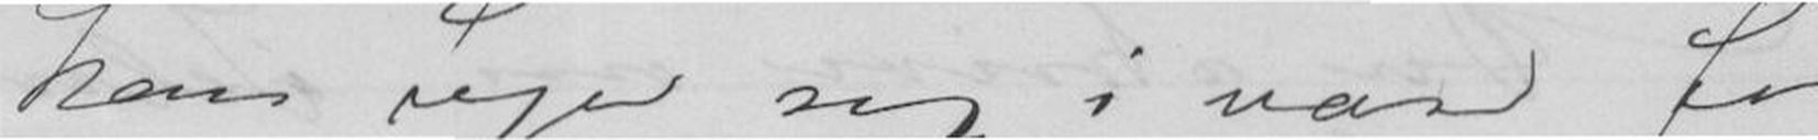han tage sig i vare for
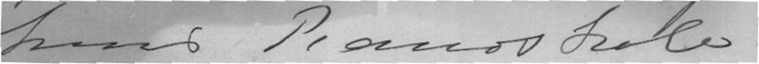hans Pianoskole
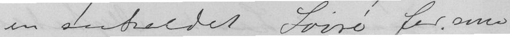en saaholdet Soiré for sine
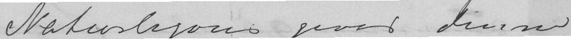Naturligvis giver denne
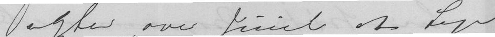agter over Juul at tage
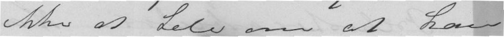ikke at tale om at han
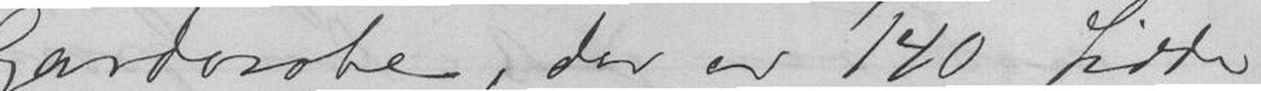Garderobe, der er 140 Sidde
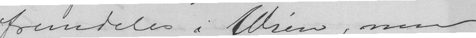fremdeles i Wien, men
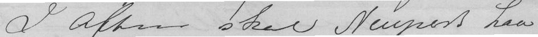I Aften skal Neupert have
